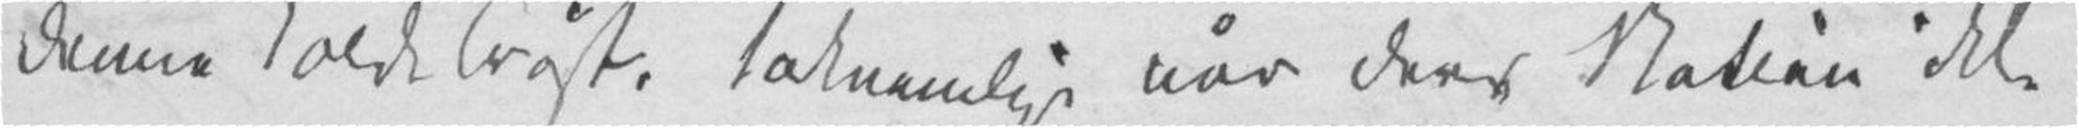denne kolde Trøst, taknemlige når deres Nation ikke
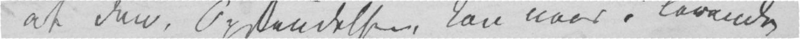at den, Opstandelsen, kan nåes i levende
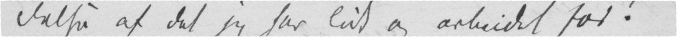delse af det jeg har lidt og arbeidet for!
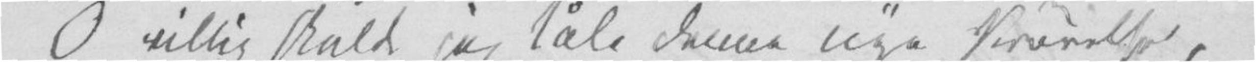O villig skulde jeg tåle denne nye Prøvelse,
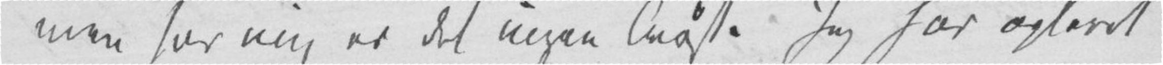men for mig er det ingen Trøst. Jeg har oplevet
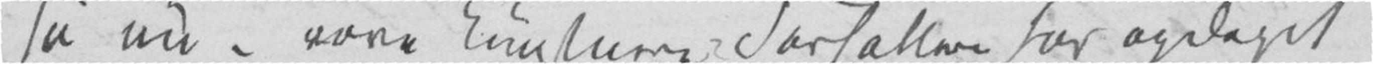så nu – vore Kunstnere, Forfattere har opdaget
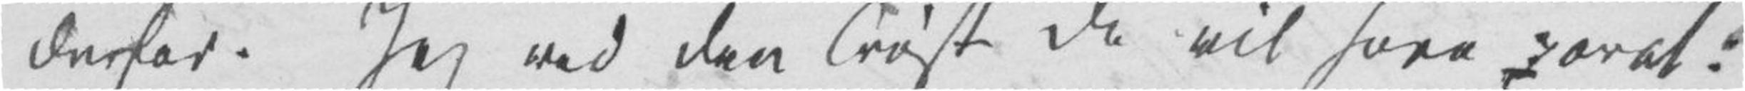derfor. Jeg ved den Trøst De vil have parat:
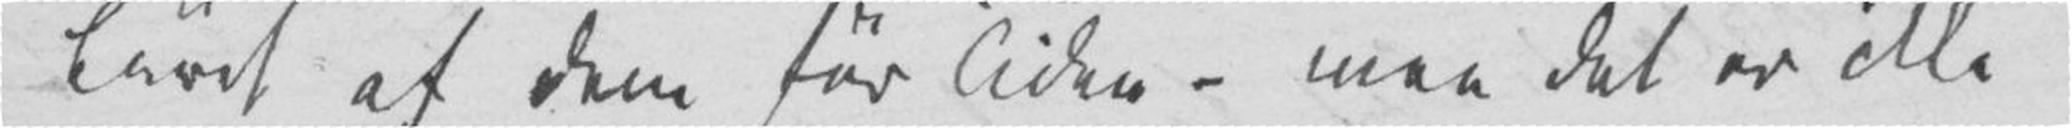Livet af dem før Tiden – men det er ikke
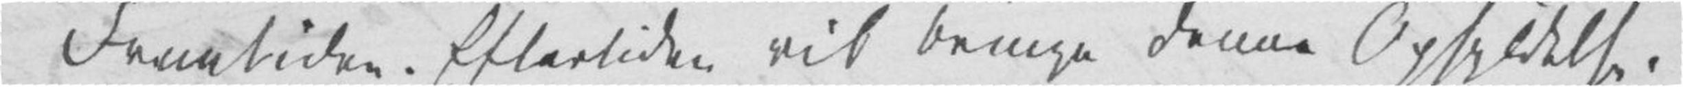«Fremtiden, Eftertiden vil bringe denne Opfyldelse[»],
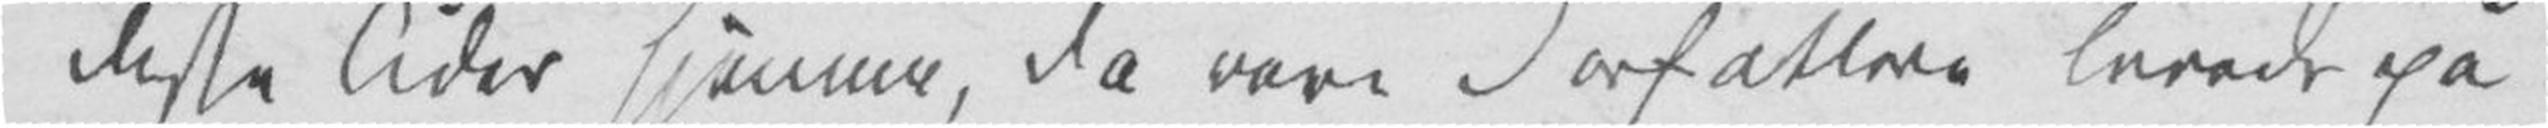disse Tider hjemme, da vore Forfattere levede på
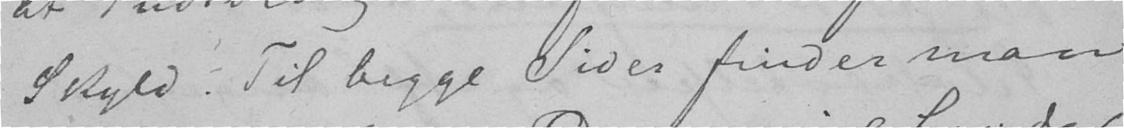Skyld. Til begge Sider finder man
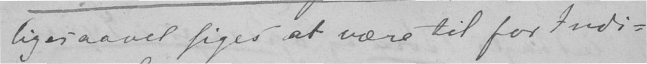ligesaavel siges at være til for Indi-
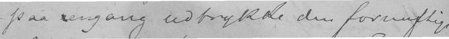paa engang udtrykke den fornuftige
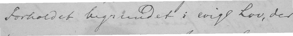Forholdet begrundet i evige Lov, der
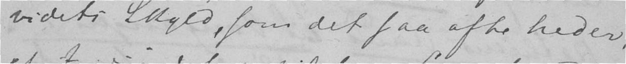videts Skyld, som det saa ofte heder,
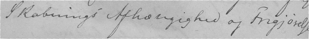Skabnings Afhængighed og Frigjørelse.
